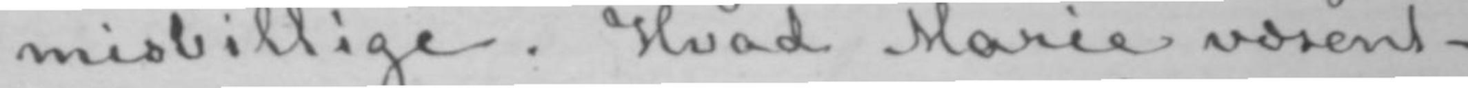misbillige. Hvad Marie væsent-
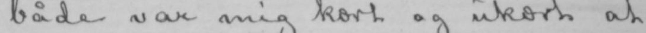både var mig kært og ukært at
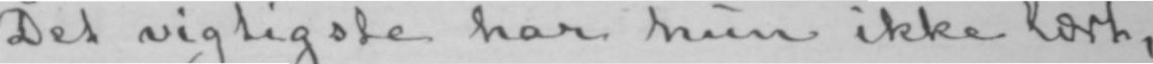Det vigtigste har hun ikke lært,
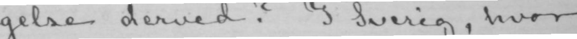gelse derved? I Sverig, hvor
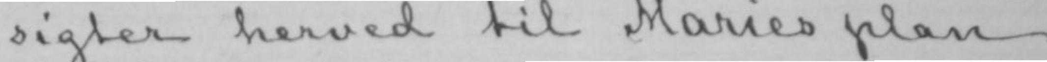sigter herved til Maries plan
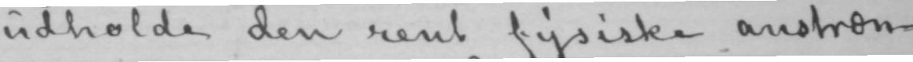udholde den rent fysiske anstræn-
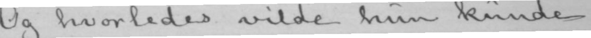Og hvorledes vilde hun kunde
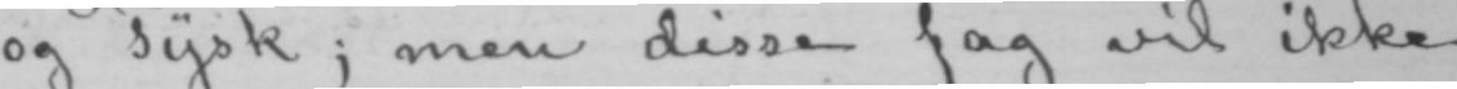og Tysk; men disse fag vil ikke
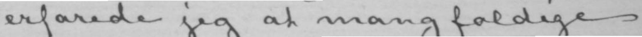erfarede jeg at mangfoldige
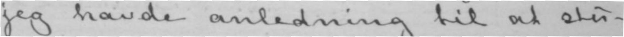jeg havde anledning til at stu-
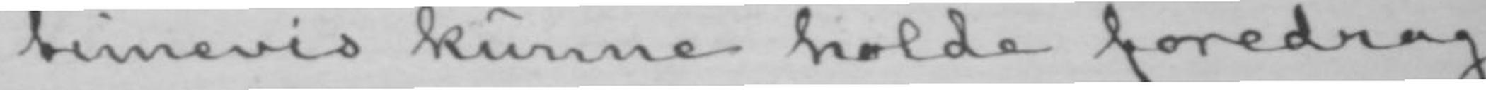timevis kunne holde foredrag
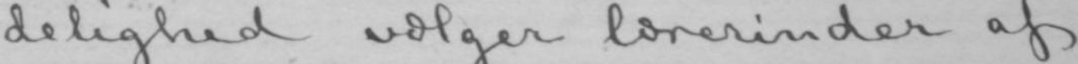delighed vælger lærerinder af
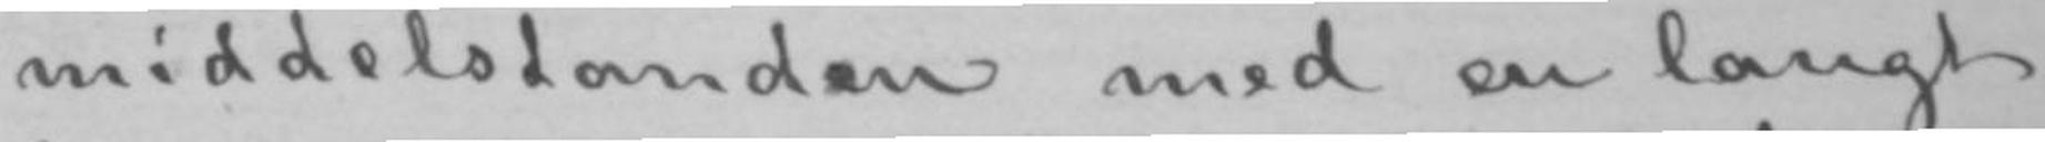middelstanden med en langt
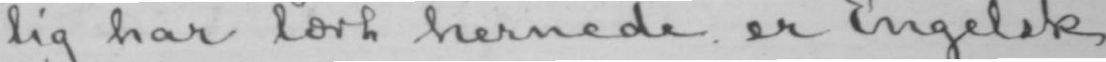lig har lært hernede er Engelsk
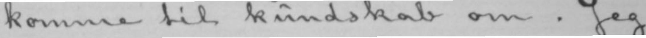komme til kundskab om. Jeg
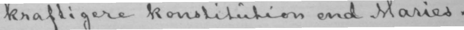kraftigere konstitution end Maries.
